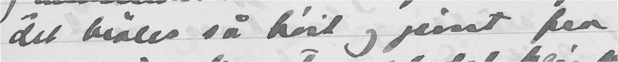det brøles så høit og jevnt fra
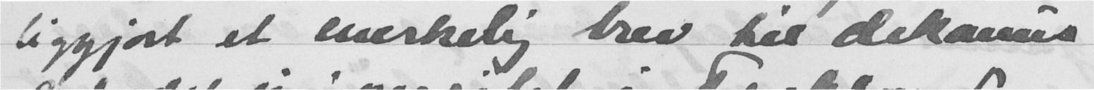liggjort et merkelig brev til dekanus
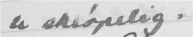er skrøpelig.
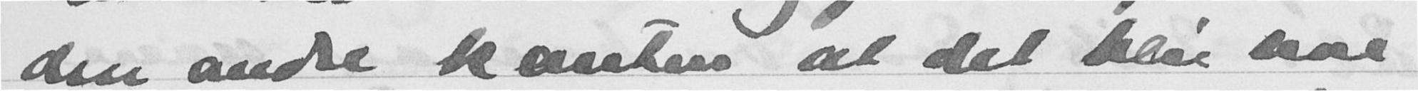den andre kanten at det blir noe
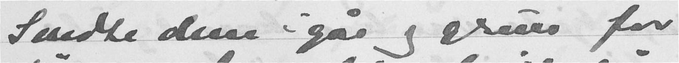Sendte dem i går og gruer for
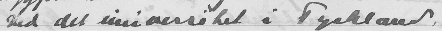ved det universitet i Tyskland,
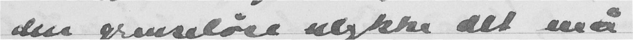den grenseløse ulykke det må
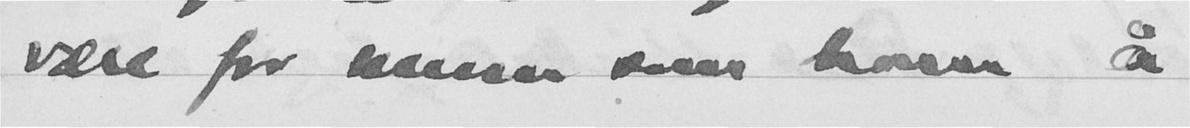være for mann som ham å
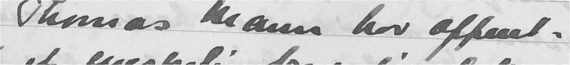Thomas Mann har offent-
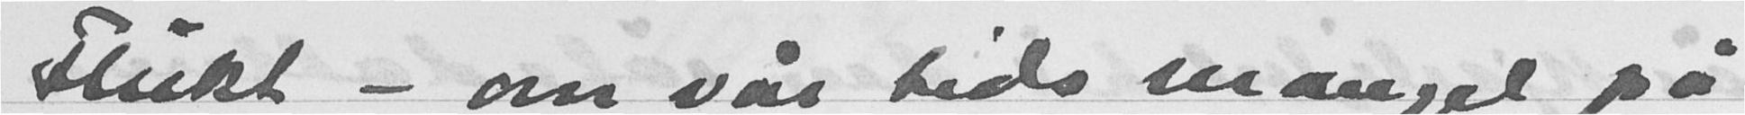flukt - om vår tids mangel på
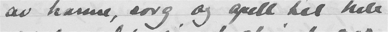av harme, sorg og apell til hele
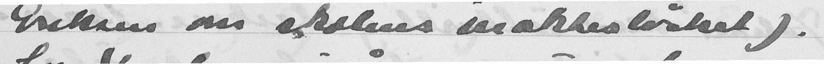Eriksen om skolens maktesløshet).
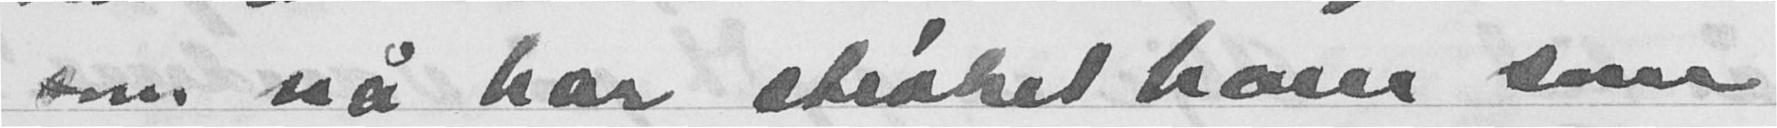som nå har strøket ham som
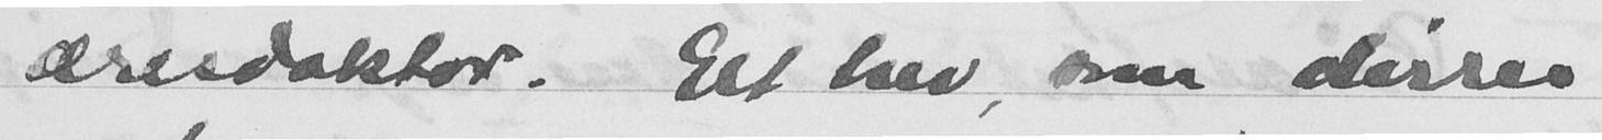æresdoktor. Et brev, som dirrer
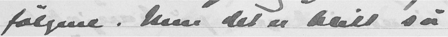følgene. Men det er blitt så
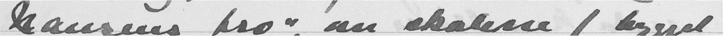Nansens "tro", om skolene (bygget
